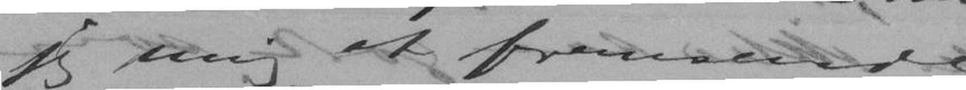jeg mig at fremsende
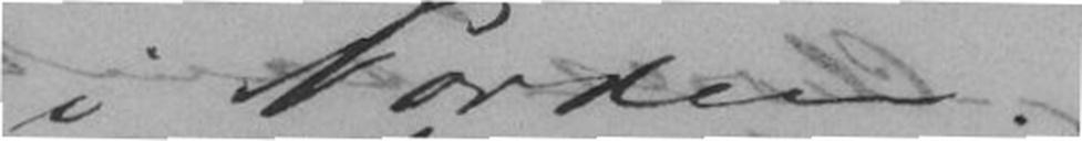i Norden.
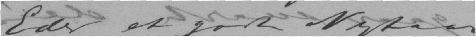Eder et godt Nytaar
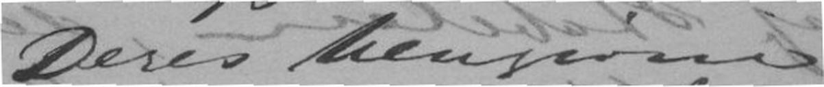Deres hengivne
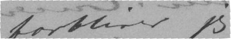forbliver jeg
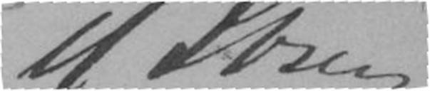H Ibsen
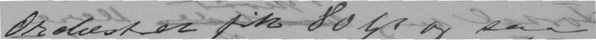Orchestret fik 80 spd og saa
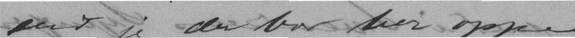end jeg der bor her oppe
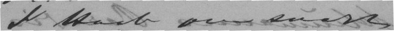I Haab om snart
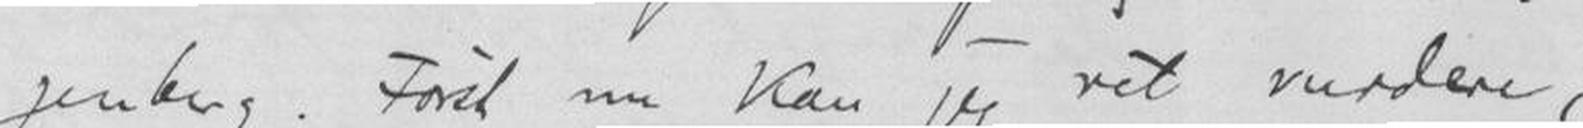genberg. Først nu kan jeg ret vurdere,
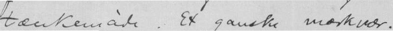tænkemåde. Et ganske mærkvær-
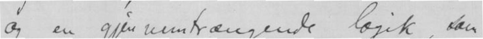og en gjennemtrængende logik, som
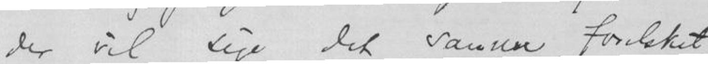der vil sige det samme, forelsket
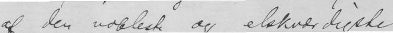af den nobleste og elskværdigste
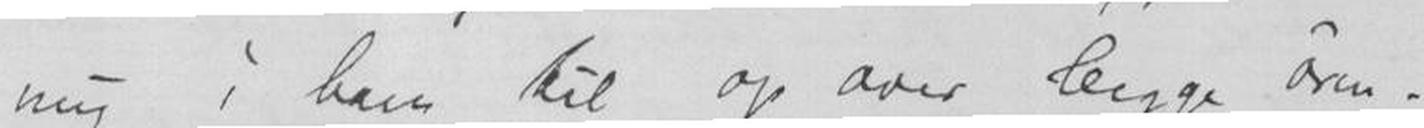mig i han til op over begge øren.
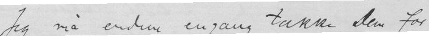Jeg må endnu engang takke Dem for
